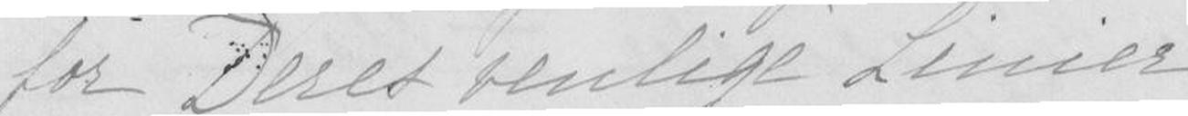for Deres venlige Linier,
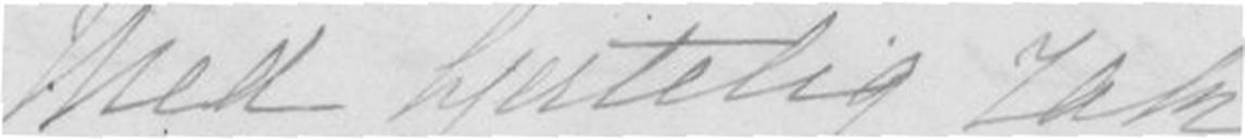Med hjertelig Tak
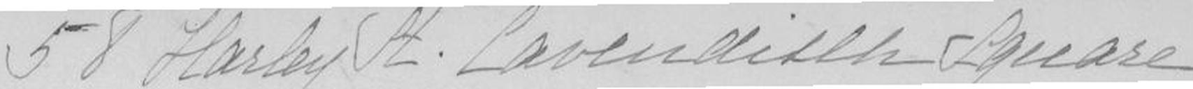58 Harley St. Carvenditch Square
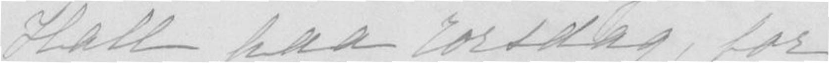Hall paa Torsdag, for
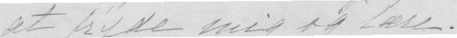at fryde mig og lære.
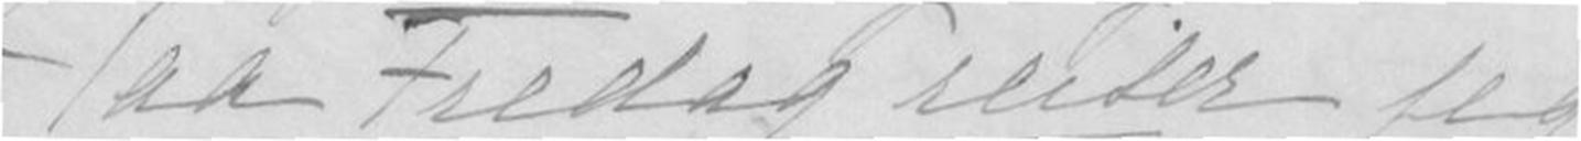Paa Fredag reiser jeg
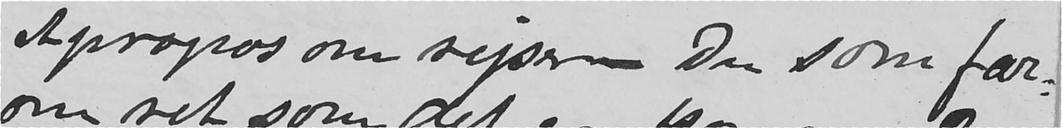Apropos om rejse- Du som far-
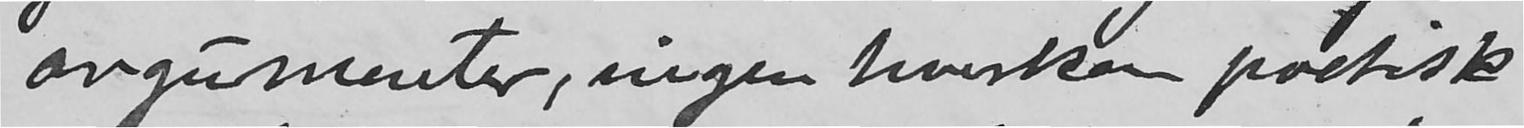argumenter, ingen hverken poetisk
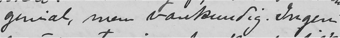genial, men vankundig. Ingen
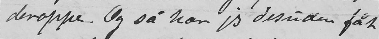deroppe. Og så har jeg desuden fåt
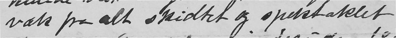væk fra alt skidtet og spektaklet
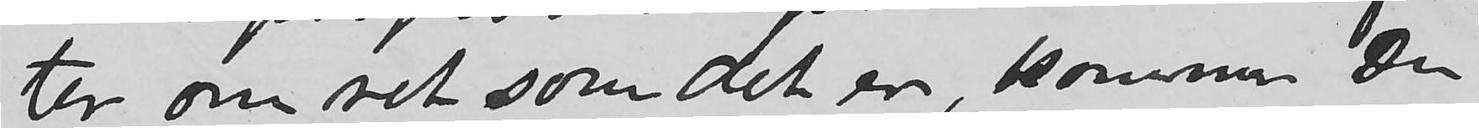ter om ret som det er, komme Du
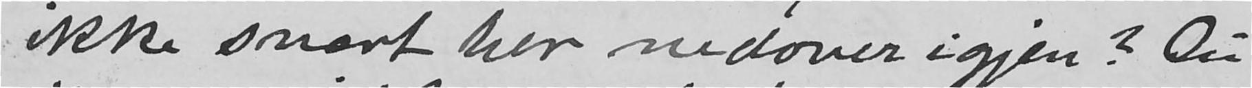ikke snart her nedover igjen? Du
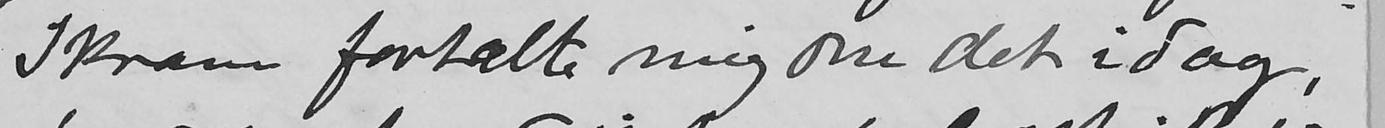Skram fortalte mig om det i dag,
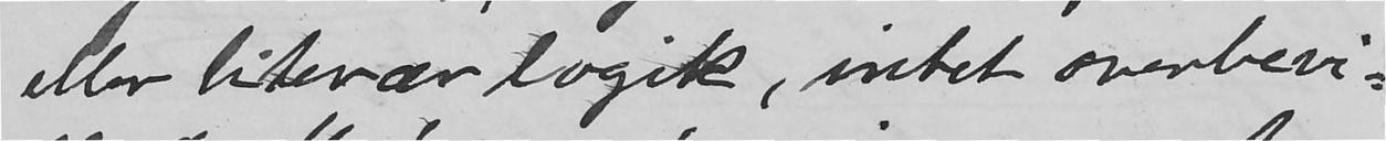eller literær logik, intet overbevi-
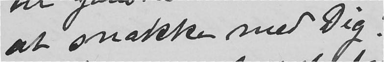at snakke med dig.
